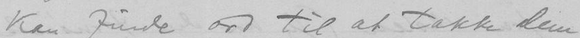kan finde ord til at takke Dem
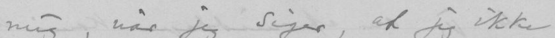mig, når jeg siger, at jeg ikke
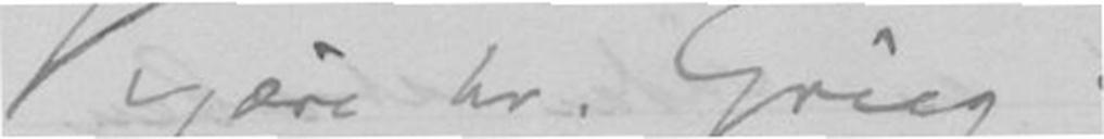Kjære hr. Grieg!
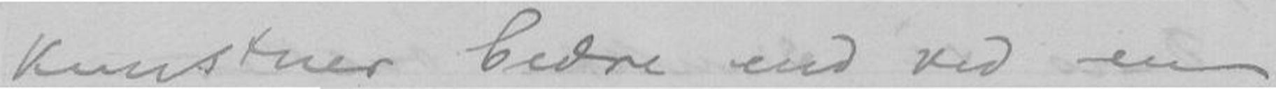kunstner bedre end ved en
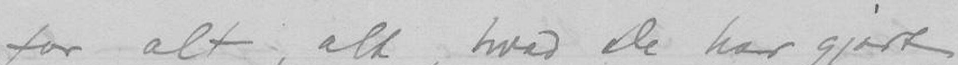for alt, alt, hvad De har gjort
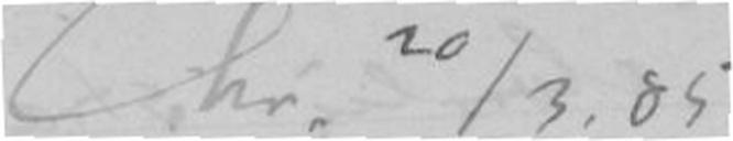Chr. 20/3.85
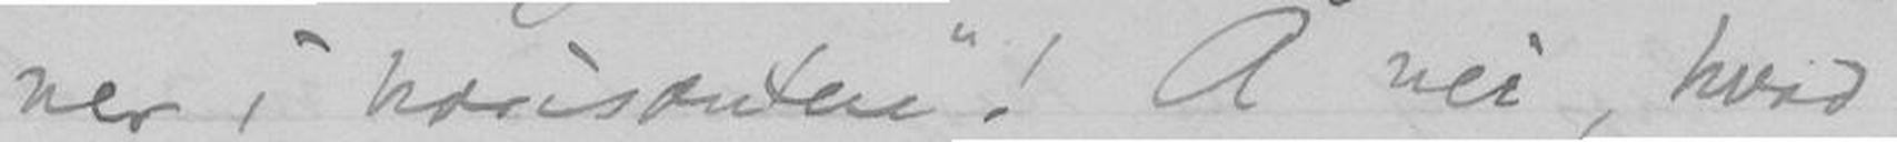ner i "horisonten"! Å nei, hvad
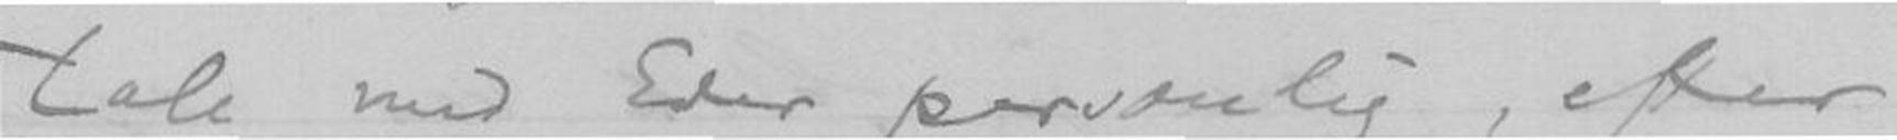tale med Eder personlig, efter
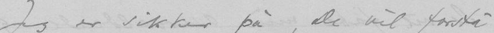Jeg er sikker på De vil forstå
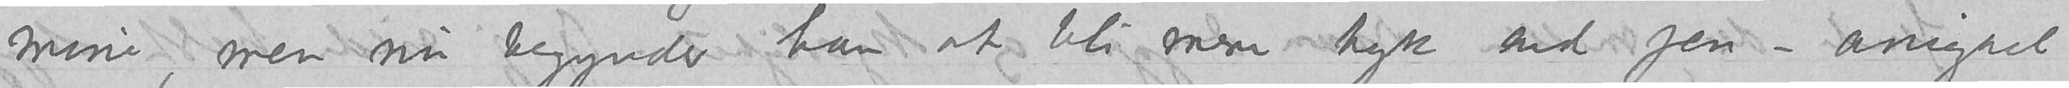mine, men nu begynder han at bli mere tyk end pen – ansigtet
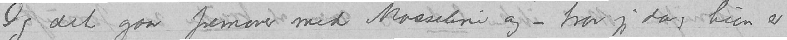Og det gaar fremover med Mosseline og – tror jeg da, hun er
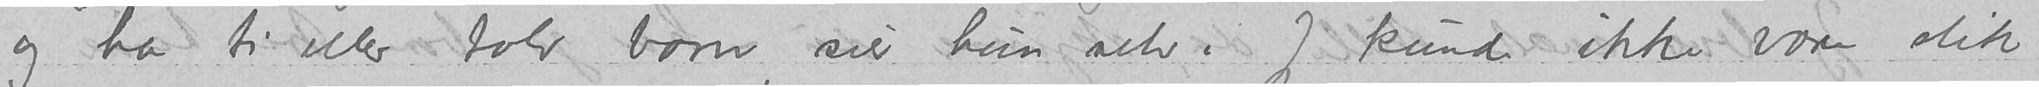og har ti eller tolv barn, sier hun selv. Jeg kunde ikke være slik
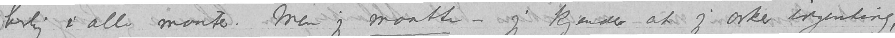herlig i alle maater. Men jeg maatte – og jeg kjender at jeg orker ingenting,
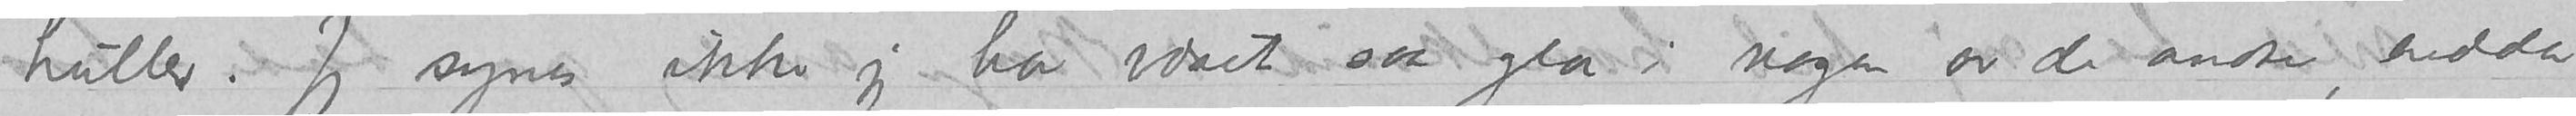huller. Jeg synes ikke jeg har været saa gla i nogen av de andre, endda
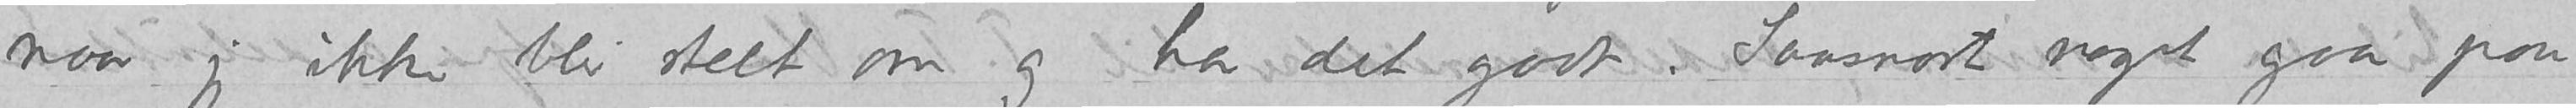naar jeg ikke blir stelt om og har det godt. Saasnart noget gaar paa
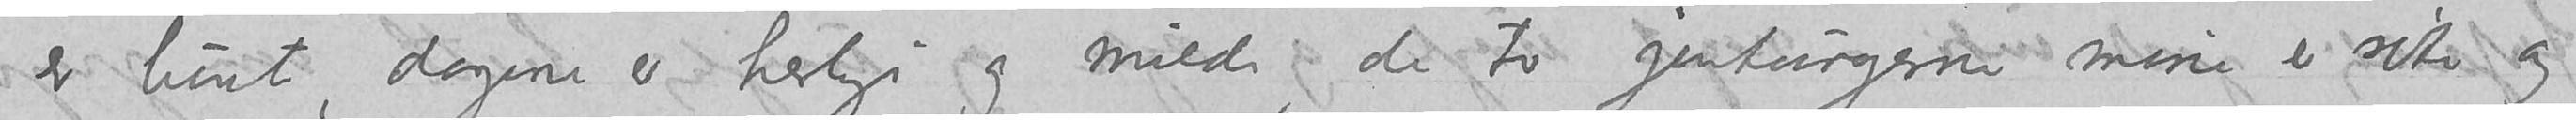er lunt, dagene er herlige og milde, de to jentungerne mine er søte og
